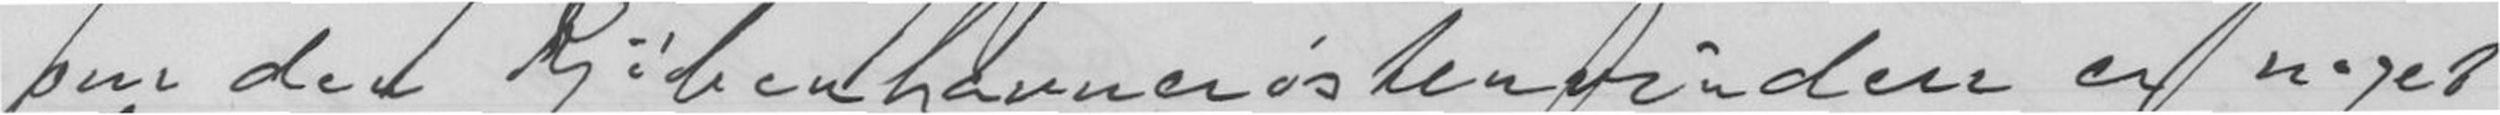om den Kjøbenhavnerøstenvinden er noget
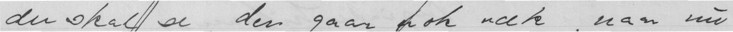du skal se, den gaar nok væk, naar nu
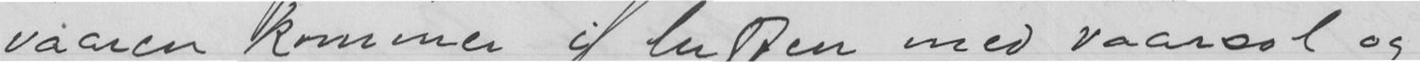vaaren kommer i luften med vaarsol og
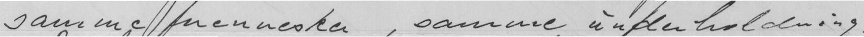samme mennesker, samme underholdning,
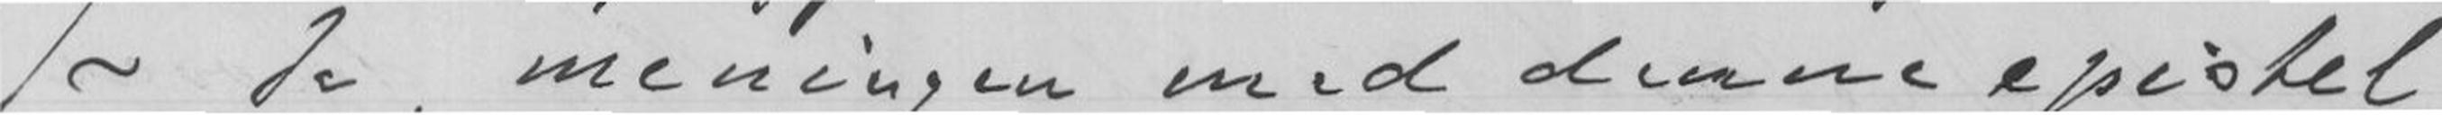- Ja, meningen med denne epistel
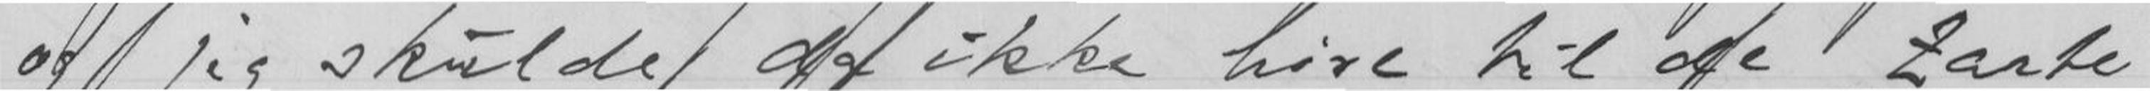og jeg skulde da ikke høre til de Zarte
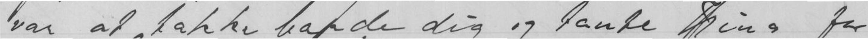var at takke baade dig og tante Nina for
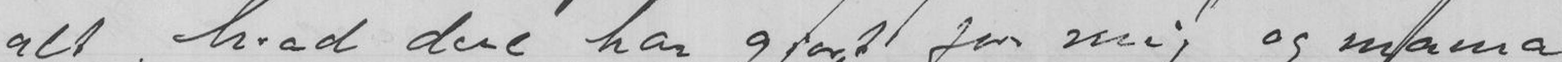alt, hvad dere har gjort for mig og mama
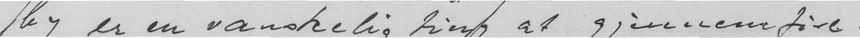by er en vanskelig ting at gjennemføre.
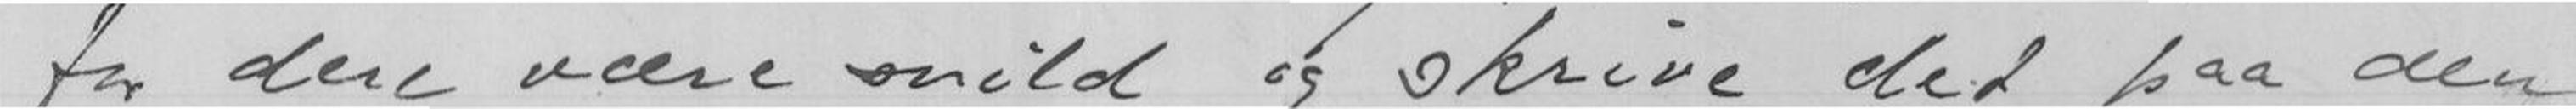for dere være snild og skrive det paa den
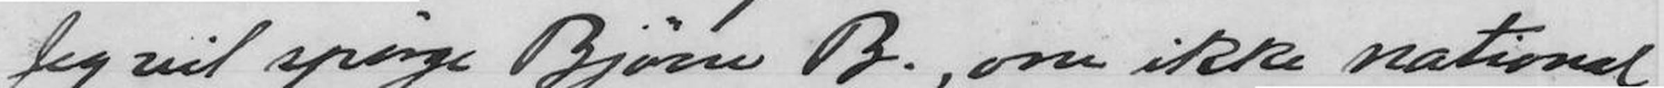Jeg vil spørge Bjørn B., om ikke national
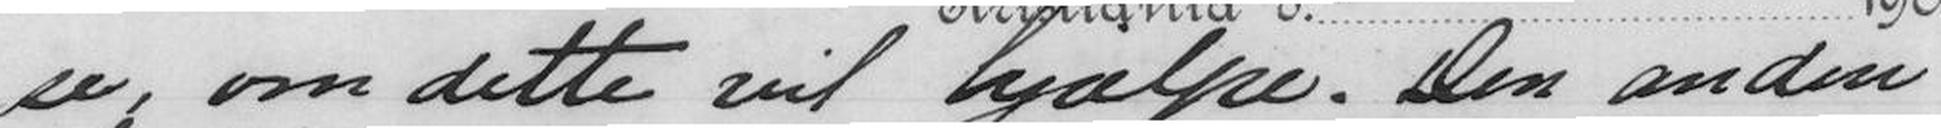se om dette vil hjælpe. Den anden
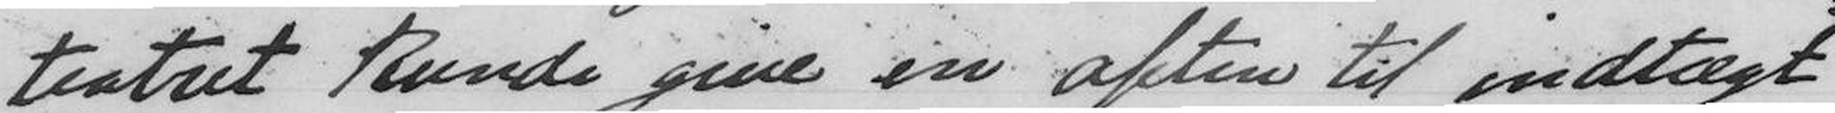teatret kunde give en aften til indtægt
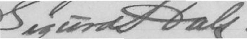Sigurd Hals.
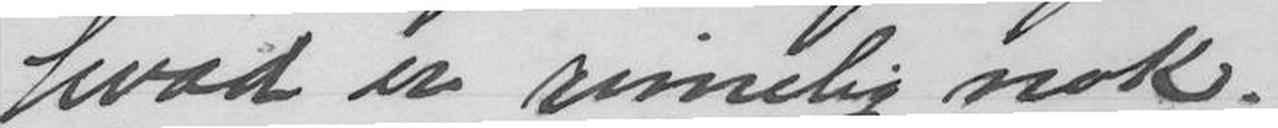hvad er rimelig nok.
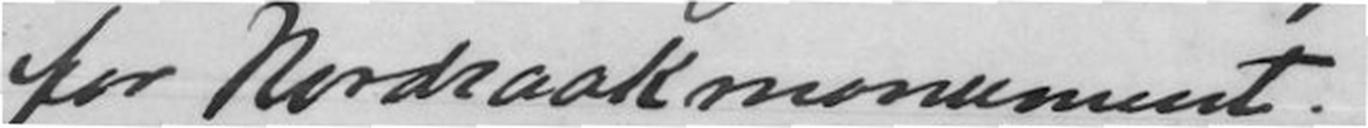for Nordraakmonument.
